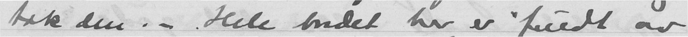tok den. - Hele bordet her er fuldt av
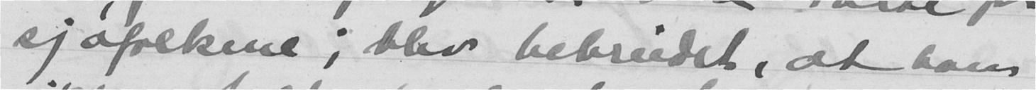sjøfolkene; blev bebreidet, at han
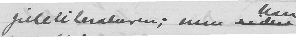juleliteraturen; men siden
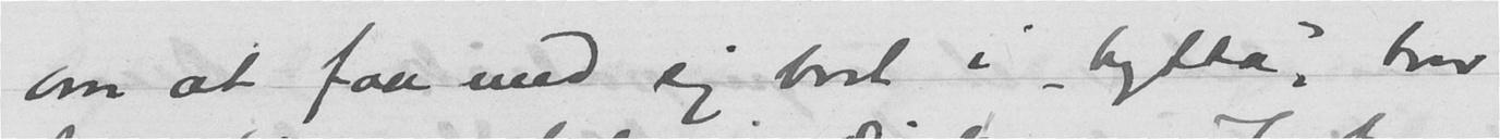om at faa med sig bort i "hytta", hvor
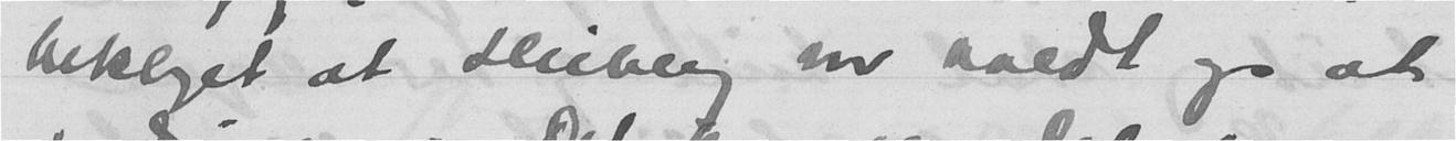beklaget at Heiberg var holdt og at
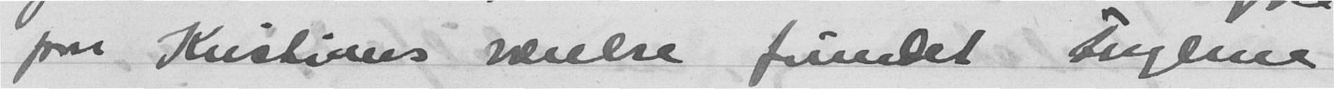paa Kristians værelse fundet Fuglene
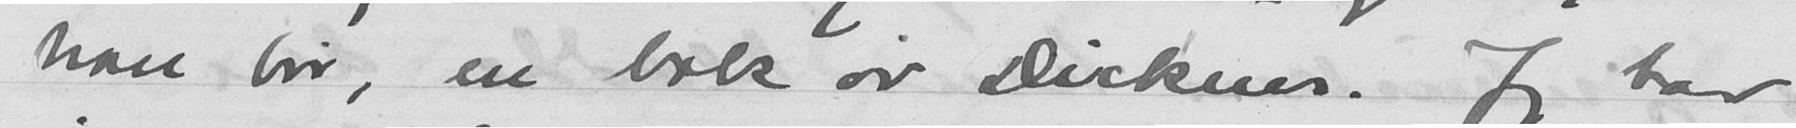han bor, en bok av Dickens. Jeg har
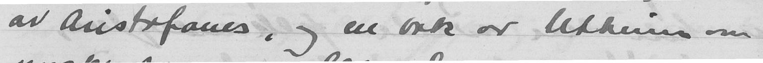av Aristofanes, og en bok av Utheims om
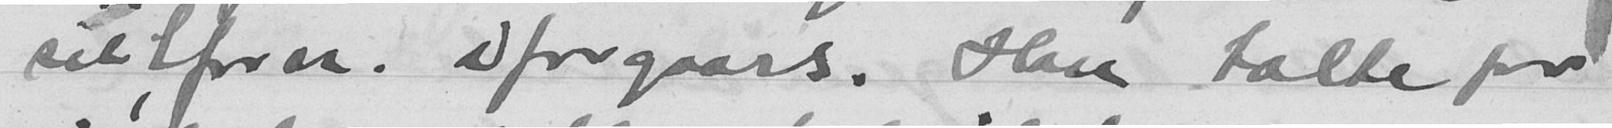seilforn. iforgaars. Han talte for
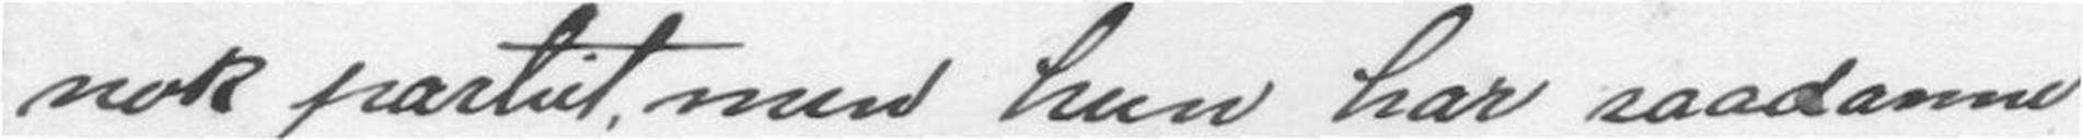nok partiet, men hun har saadanne
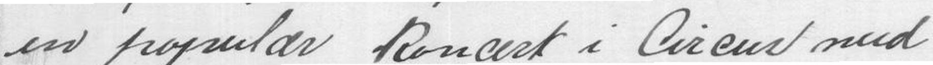en populær koncert i Circus med
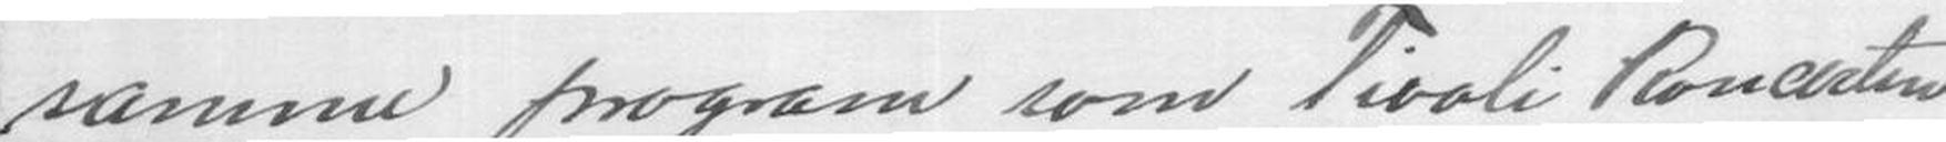samme program som Tivoli koncerten
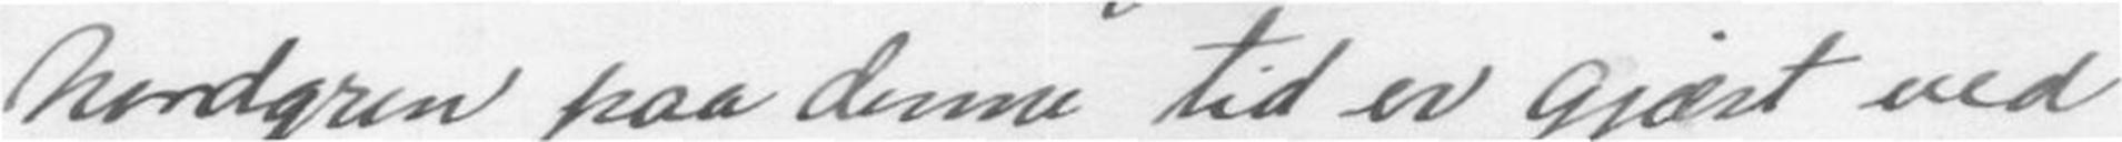Nordgren paa denne tid er gjæst ved
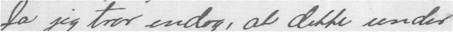Ja jeg tror endog, at dette under
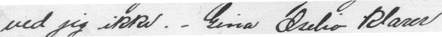ved jeg ikke. - Gina Oselio klarer
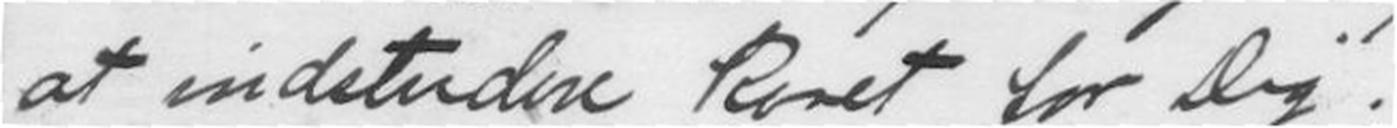at indstudere Koret for Dig.
